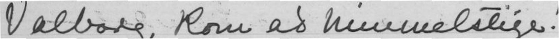Valborg, kom ad himmelstige!
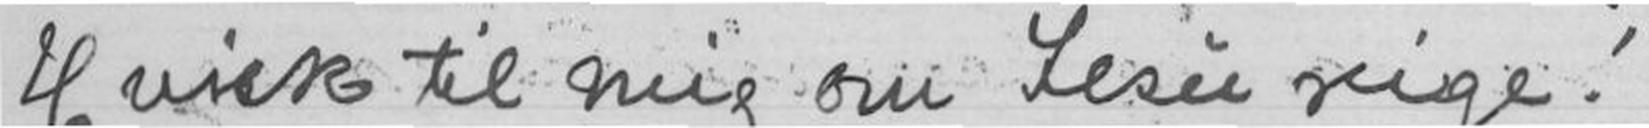Hvisk til mig om Jesu rige!
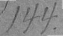144.
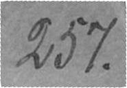257.
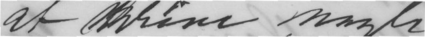at skrive nogle
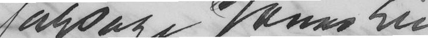forsøge Janns liv
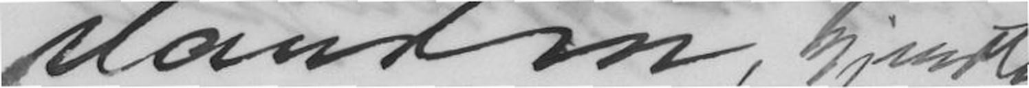Manden, kjendte
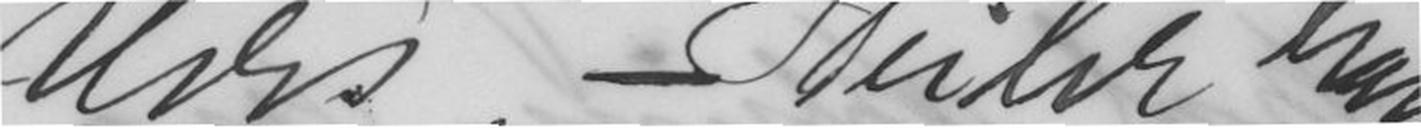Værs. - Steiler han
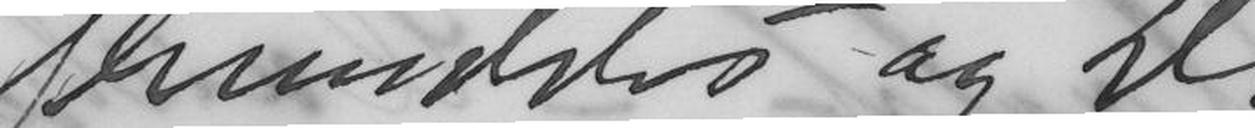fremdeles og De
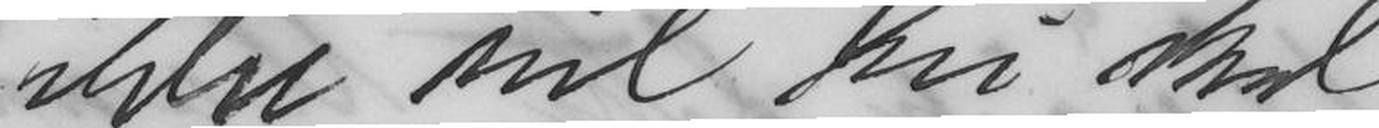ikke vil vi skal
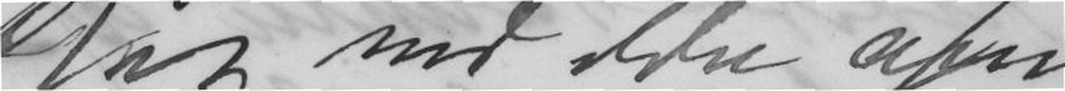Jeg ved ikke om
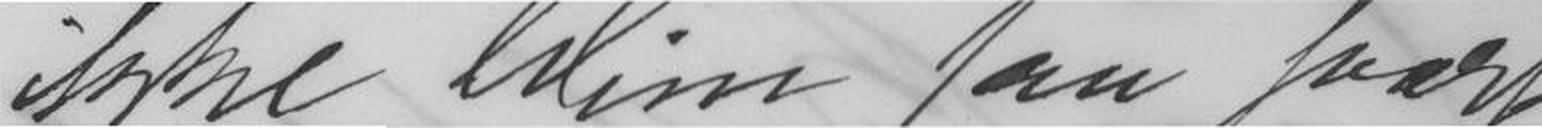ikke blive saa svært
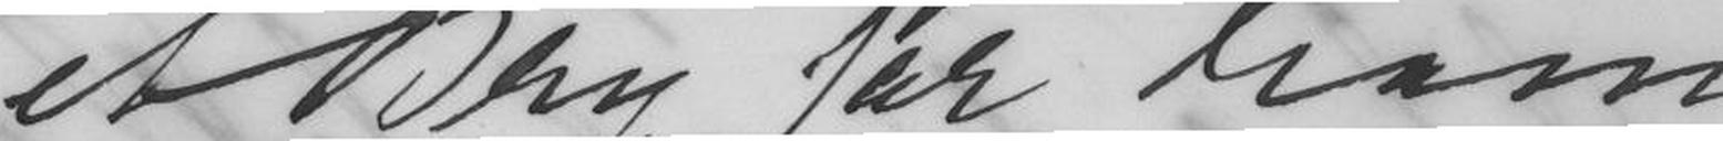et Bry for ham
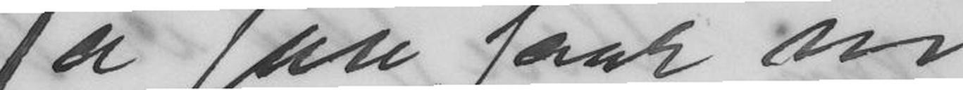ja saa faar vi
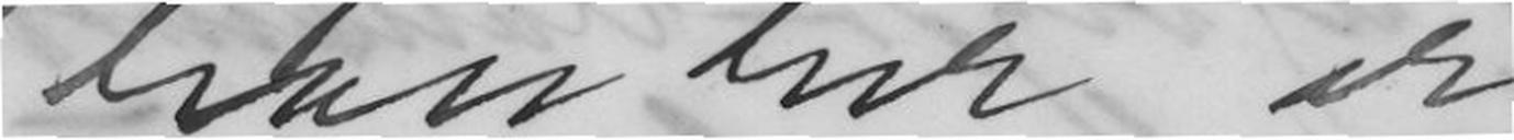han her er
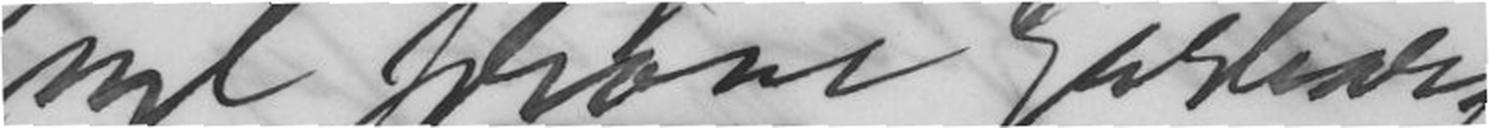vel prøve Garborg.
